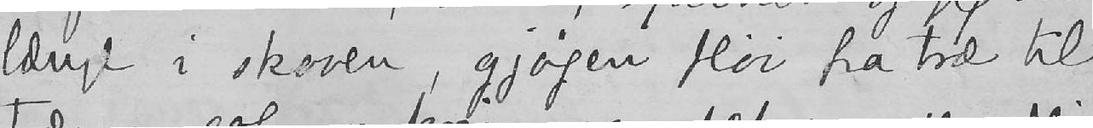længe i skoven, gjøgen fløi fra træ til
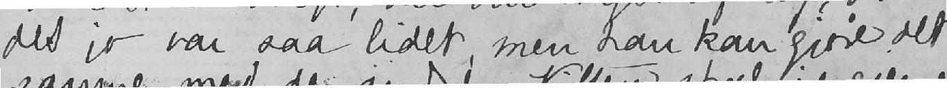det jo var saa lidet, men han kan gjøre det
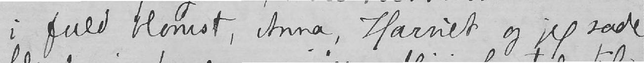i fuld blomst, Anna, Harriet og jeg sade
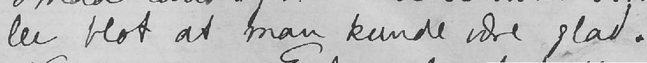ler blot at man kunde være glad.
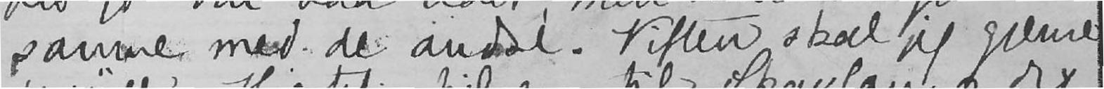samme med de andre. Viften skal jeg gjerne
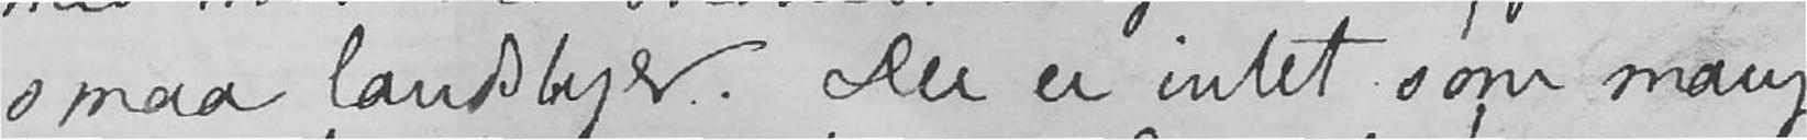smaa landsbyer. Der er intet som mang-
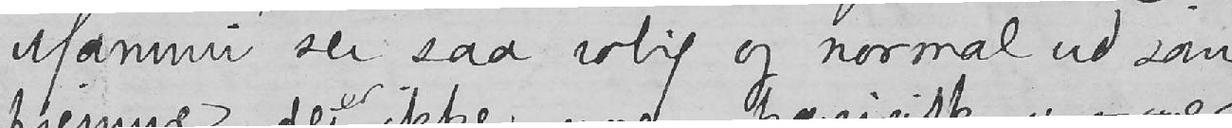Manni ser saa rolig og normal ud som
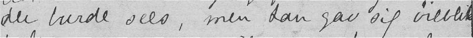der burde sees, men han gav sig øieblik-
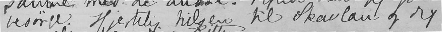besørge. Hjertelig hilsen til Skavlan og dig
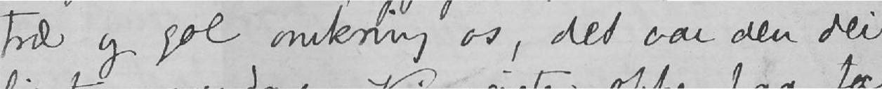træ og gol omkring os, det var den dei-
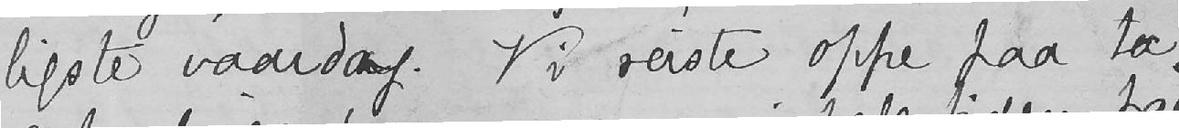ligste vaardag. Vi reiste oppe paa ta-
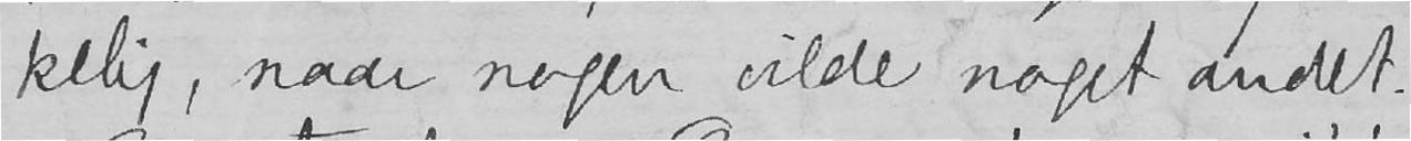kelig, naar nogen vilde noget andet.
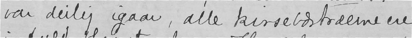var deilig igaar, alle kirsebærtræerne ere
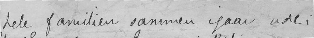hele familien sammen igaar, ude i
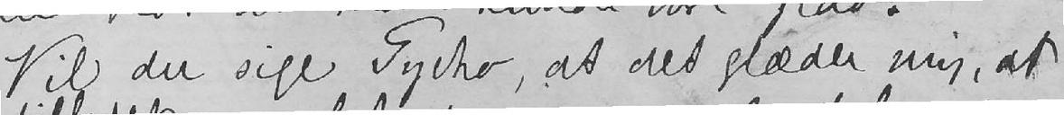Vil du sige Tycho, at det glæder mig, at
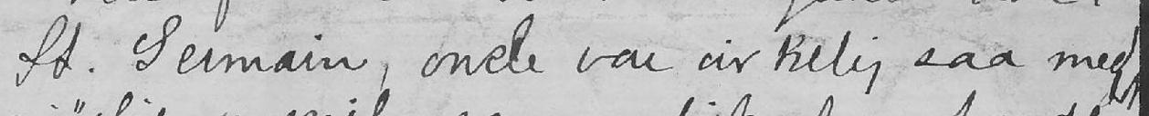St. Germain, oncle var virkelig saa med-
